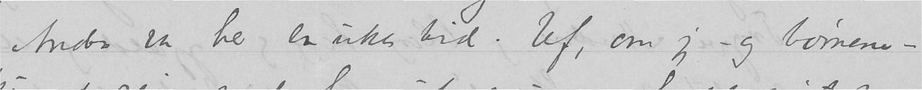Anders var her en ukes tid. Uf, om jeg – og børnene –
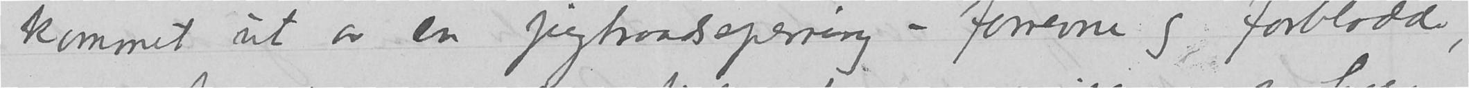kommet ut av en pigtraadssperring – forrevne og forblødde
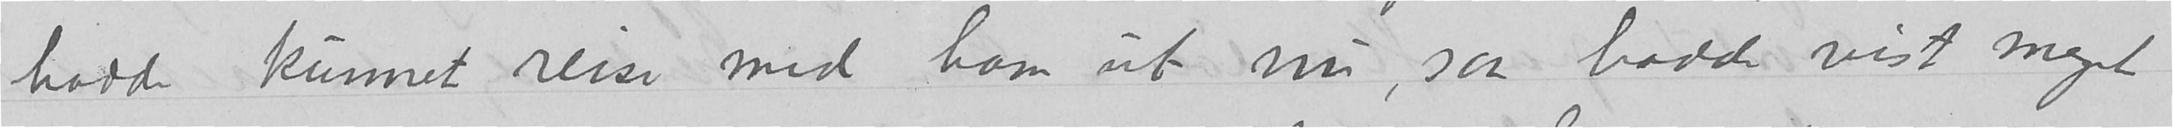hadde kunnet reise med ham ut nu, saa hadde vist meget
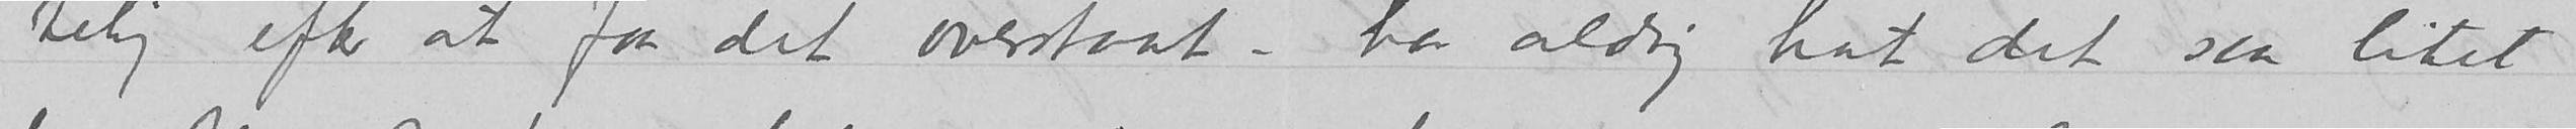telig efter at faa det overstaat – har aldrig hat det saa litet
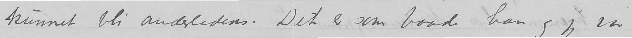kunnet bli anderledens. Det er som baade han og jeg var
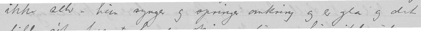ikke selv – hun synger og springer omkring og er gla og det
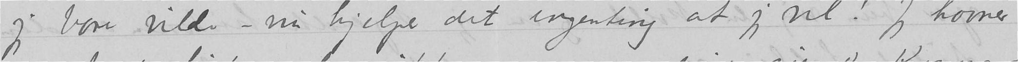jeg bare vilde – nu hjelper det ingenting at jeg vil! Jeg hovner
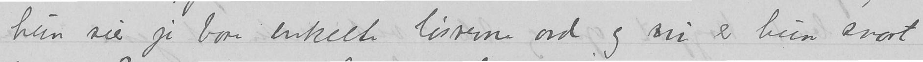hun sier jo bare enkelte løsnerne ord og nu er hun snart
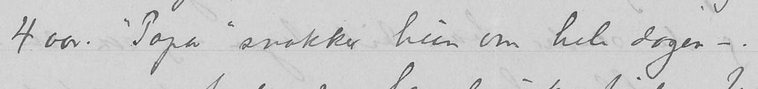4 aar. Papa snakker hun om hele dagen –.
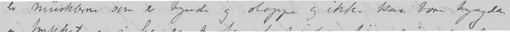er musklerne som er tynde og slappe og ikke kan bære tyngden
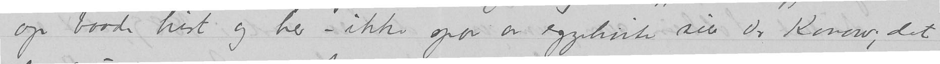op baade hist og her – ikke spor av eggehvite sier Dr. Konow, det
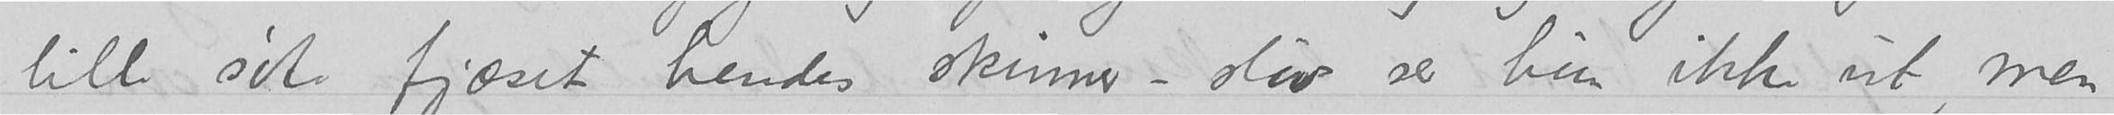lille søte fjæset hendes skinner – sløv ser hun ikke ut, men
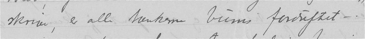skrive, er alle tankerne bums forduftet –.
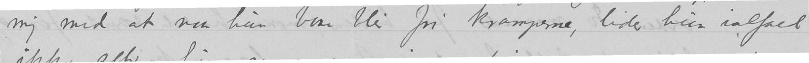mig med at naar hun bare blir fri kramperne, lider hun ialfald
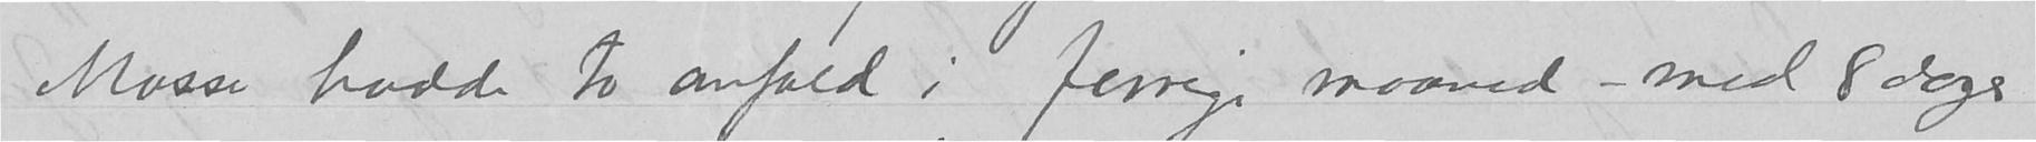Mosse hadde to anfald i forrige maaned – med 8 dages
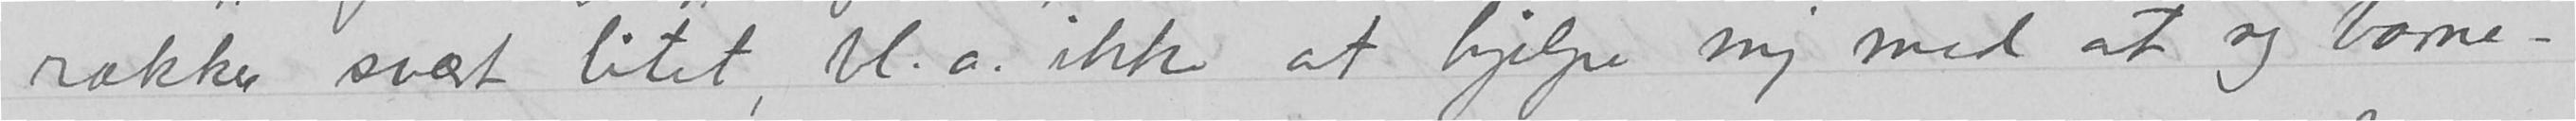rækker svært litet, bl.a. ikke at hjelpe mig med at sy barne –
